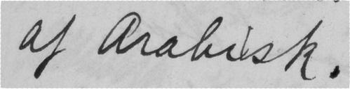af Arabisk.
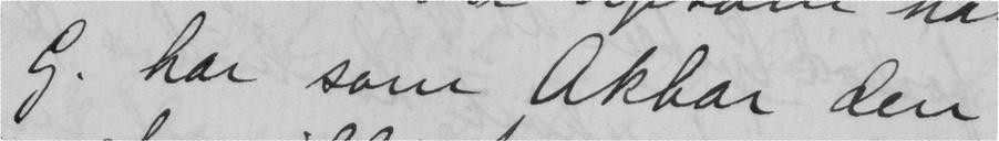G. har som Akbar den
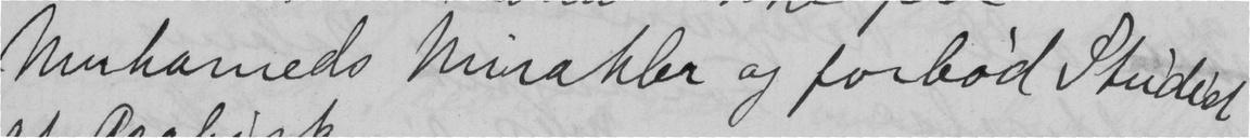Muhameds mirakler og forbød Studiet
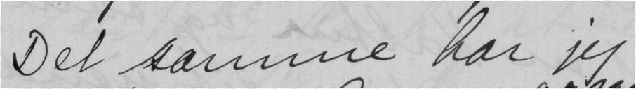Det samme har jeg
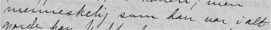menneskelig som han var i alt
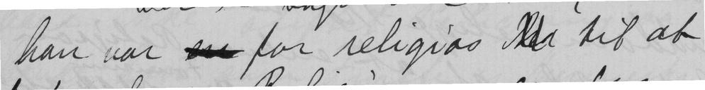han var  for religiøs  til at
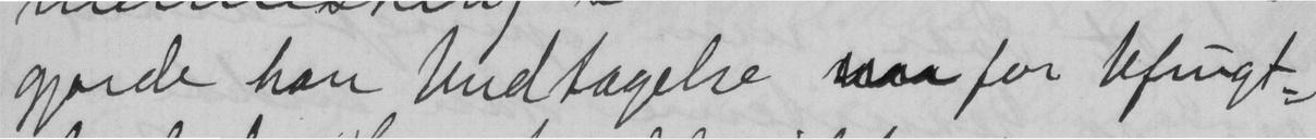gjorde han Undtagelse  for Ufrugt-
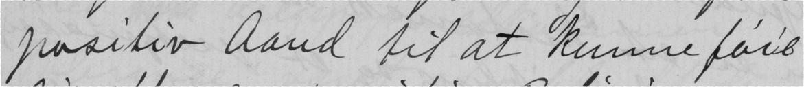positiv Aand til at kunne føie
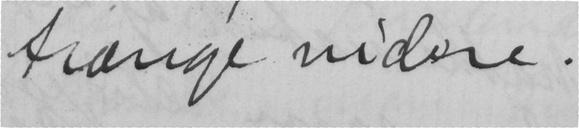trænge videre.
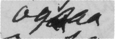ogsaa
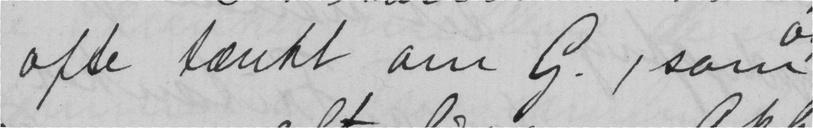ofte tænkt om G., som
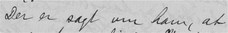Der er sagt om ham, at
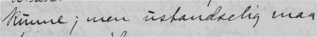kunne; men ustandselig maa
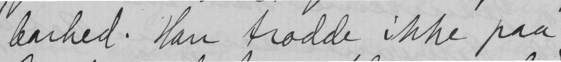barhed. Han trodde ikke paa
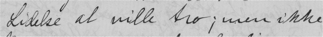Lidelse at ville tro; men ikke
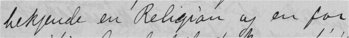bekjende en Religion og en for
